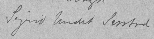Sigrid Undset Svarstad
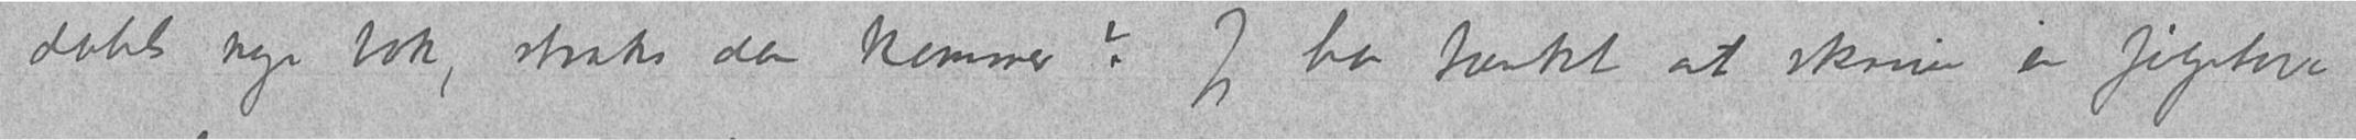dahls nye bok, straks den kommer? Jeg har tænkt at skrive en føljeton
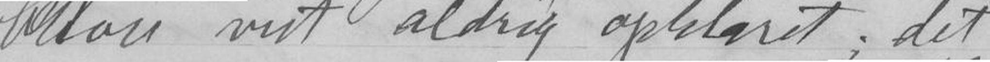blive vist aldrig opklaret; det
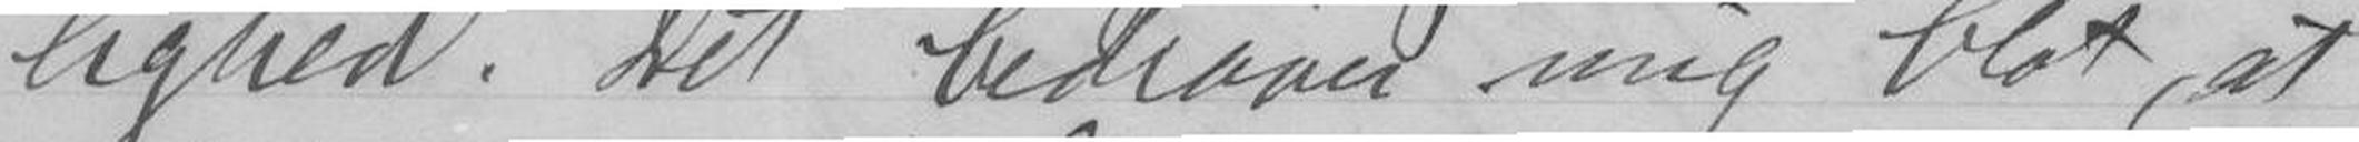lighed. Det bedrøver mig blot, at
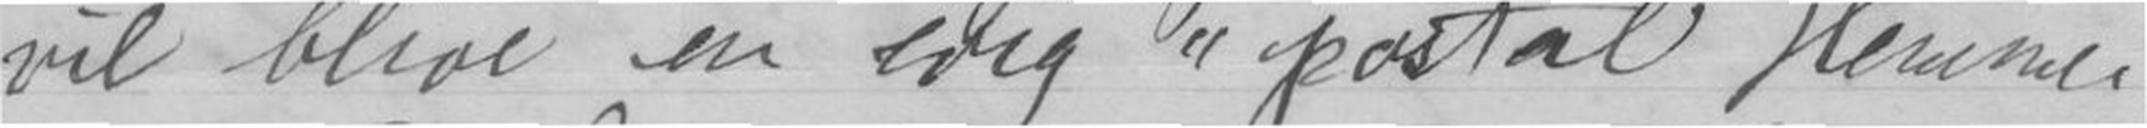vil blive en evig postal Hemme-
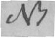NB
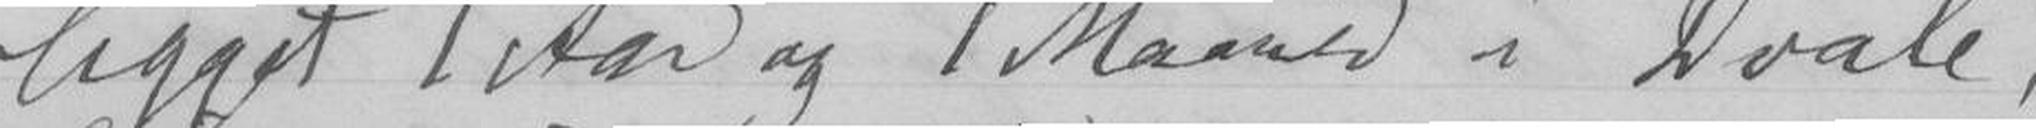ligget 1 Aar og 1 Maaned i Dvale,
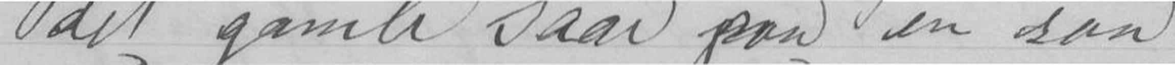det gamle Saar paa en saa
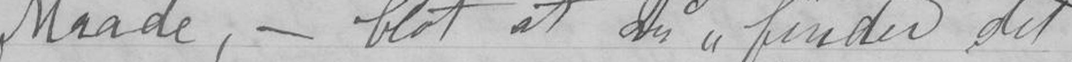Maade, - blot at Du finder det;
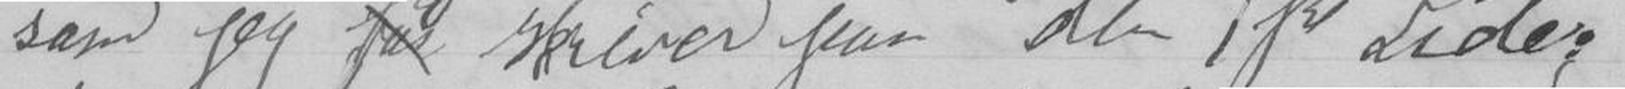som jeg skriver paa den 1ste Side;
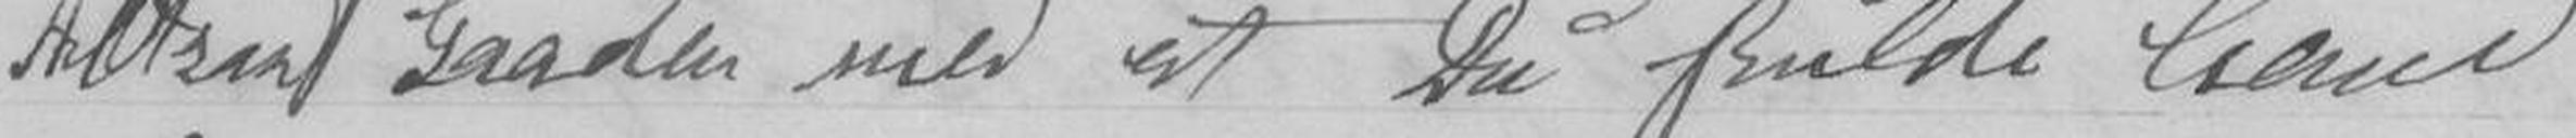Altsaa Gaaden med at Du skulde have
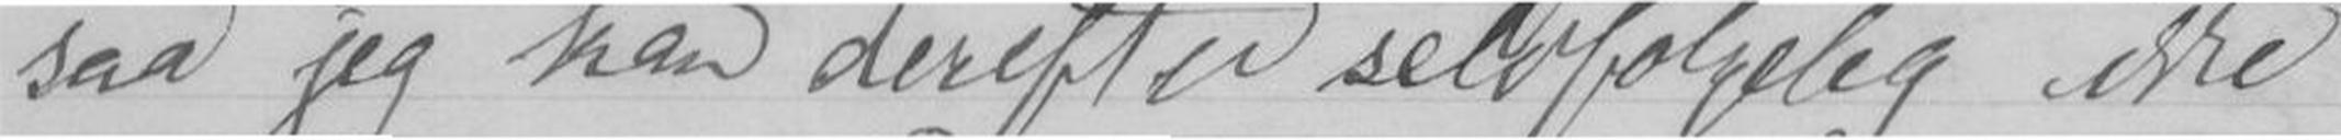saa jeg kan derefter selvfølgelig ikke
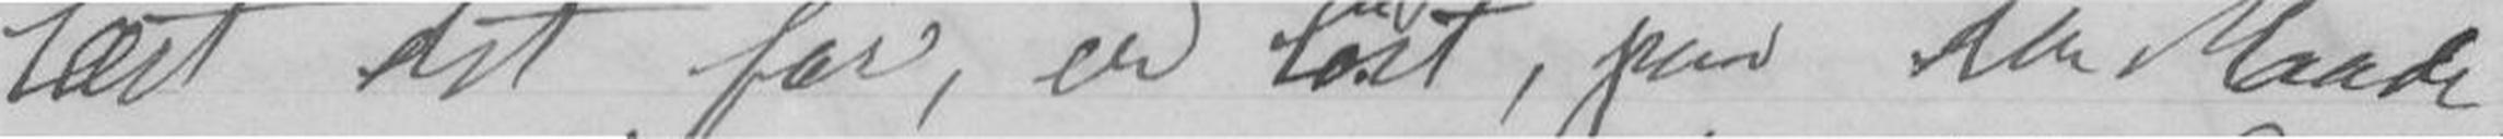læst det før, er løst, paa den Maade
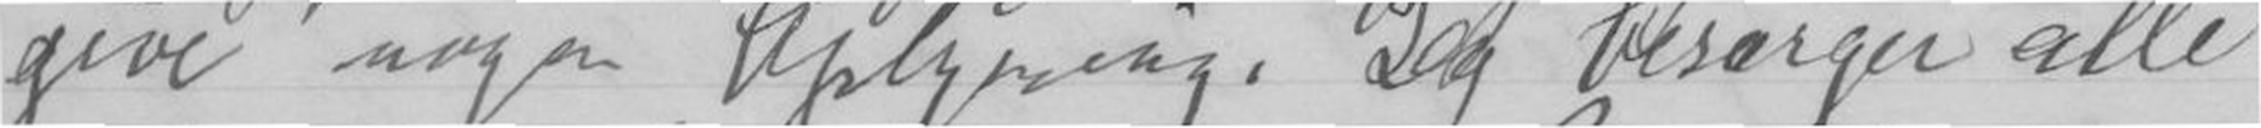give nogen Oplysning. Jeg besørger alle
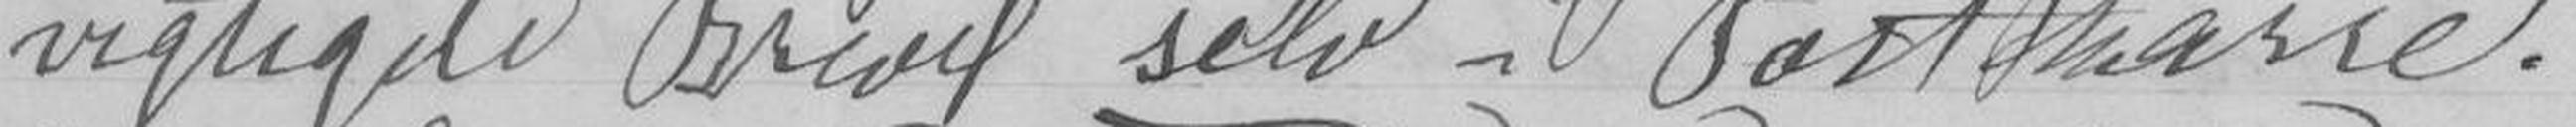vigtigere Breve selv i Postkasse.
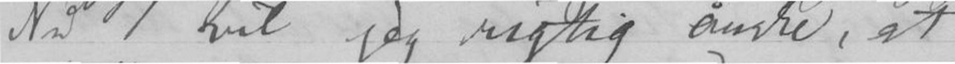Nu vil jeg rigtig ønske, at
